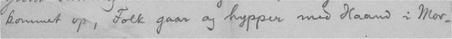kommet op, Folk gaar og hypper med Haand i Mor-
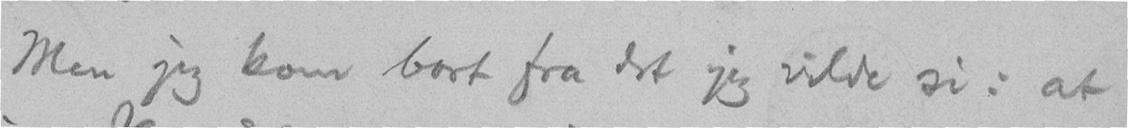Men jeg kom bort fra det jeg vilde si: at
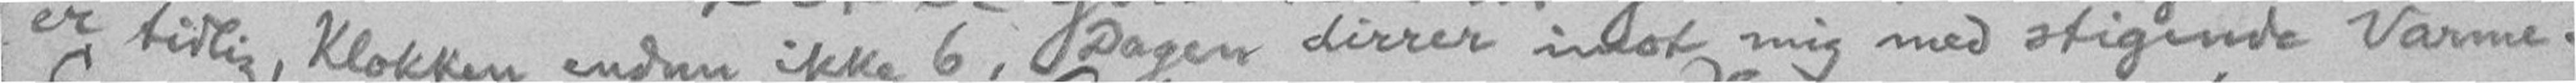er tidlig, Klokken endnu ikke 6, Dagen dirrer imot mig med stigende Varme.
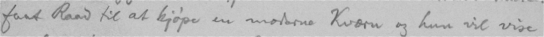faat Raad til at kjøpe en moderne Kværn og hun vil vise
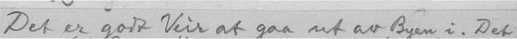Det er godt Veir at gaa ut av Byen i. Det
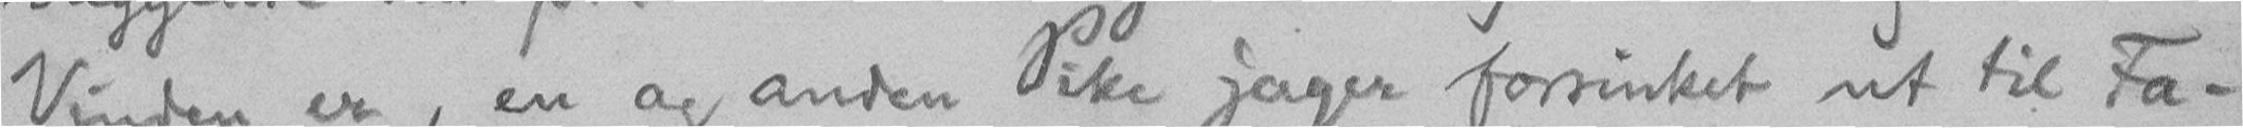Vinden er, en og anden Pike jager forsinket ut til Fa-
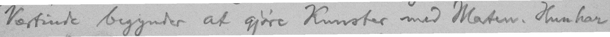Værtinde begynder at gjøre Kunster med Maten. Hun har
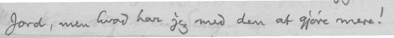Jord, men hvad har jeg med den at gjøre mere!
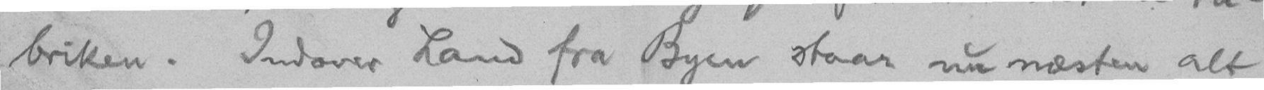briken. Indover Land fra Byen staar nu næsten alt
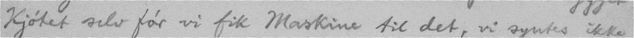Kjøtet selv før vi fik Maskine til det, vi syntes ikke
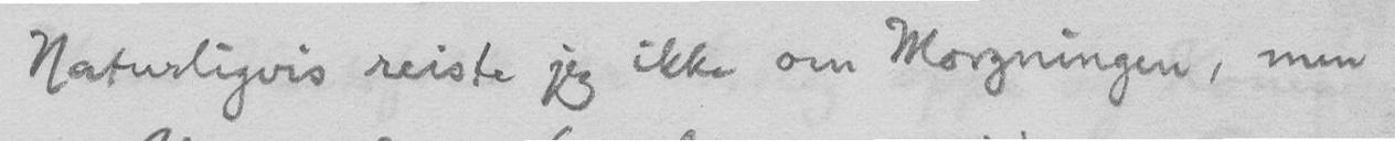Naturligvis reiste jeg ikke om Morgningen, men
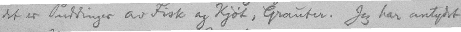det er Puddinger av Fisk og Kjøt, Grauter. Jeg har antydet
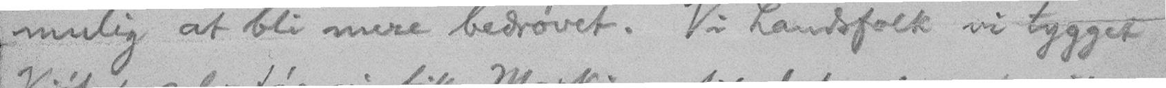mulig at bli mere bedrøvet. Vi Landsfolk vi tygget
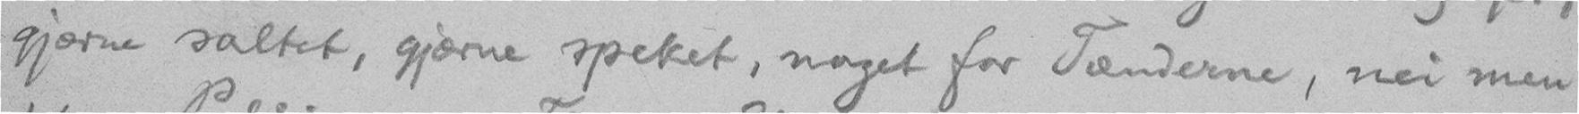gjærne saltet, gjærne speket, noget for Tænderne, nei men
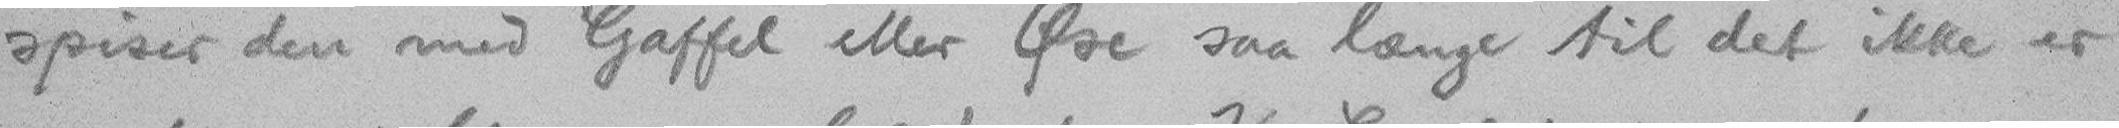spiser den med Gaffel eller Øse saa længe til det ikke er
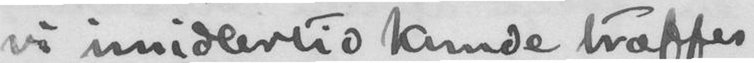vi imidlertid kunde træffes
